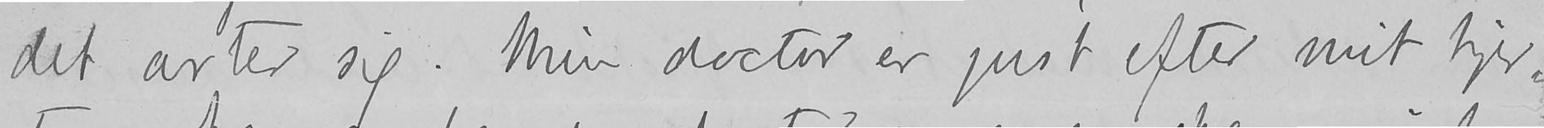det arter sig. Min doctor er just efter mit hjer-
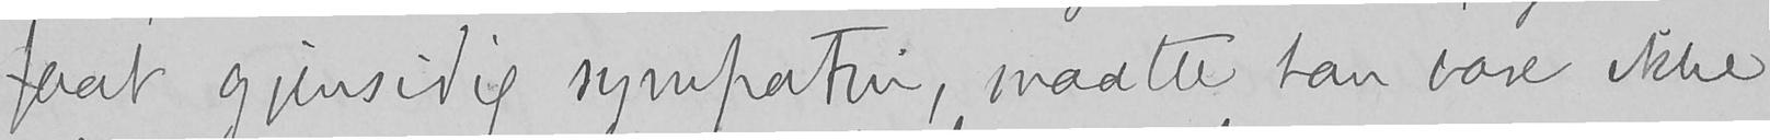faat gjensidig sympathi, maatte han bare ikke
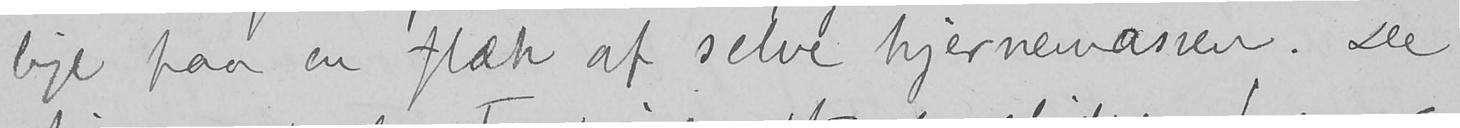lige paa en flæk af selve hjernemassen. De
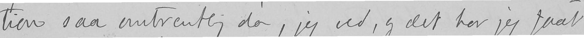tion saa omtrentlig da, jeg ved, og det har jeg faat
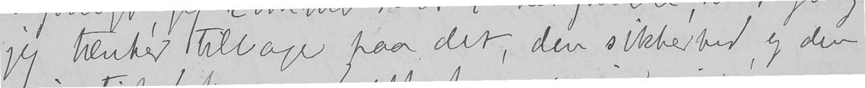jeg tænker tilbage paa det, den sikkerhed, og den
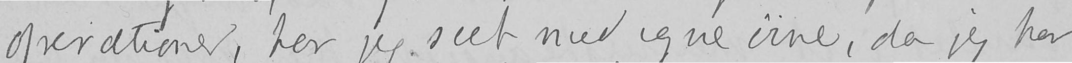operationer, har jeg seet med egne øine, da jeg har
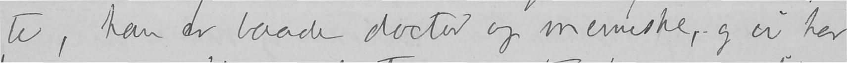te, han er baade doctor og menneske, og vi har
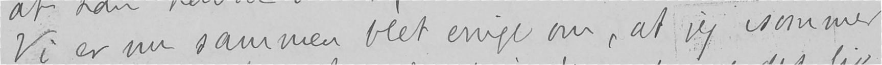Vi er nu sammen blet enige om, at jeg isommer
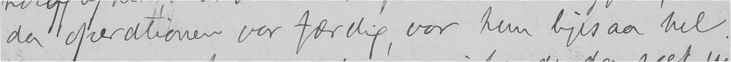da operationen var færdig, var hun ligesaa hel
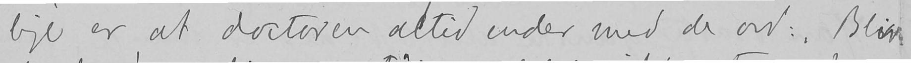lige er, at doctoren altid ender med de ord: Bliver
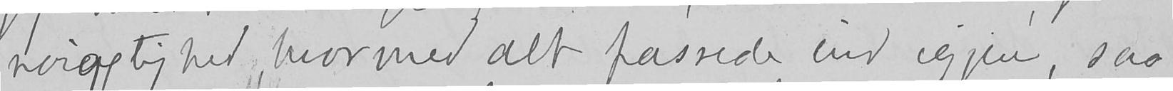nøiagtighed, hvormed alt passede ind igjen, saa
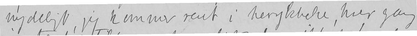nydeligt, jeg kommer rent i henrykkelse, hver gang
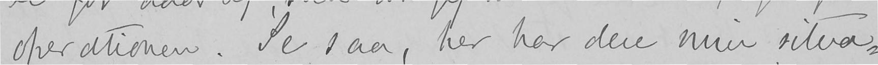operationen. Se saa, her har dere min situa-
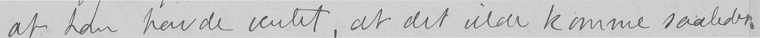at han havde ventet, at det vilde komme saaledes.
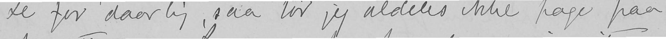de for daarlig saa tør jeg aldeles ikke tage paa
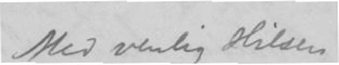Med venlig Hilsen
